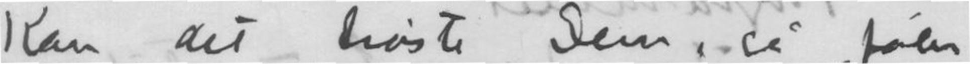Kan det trøste Dem. så føler
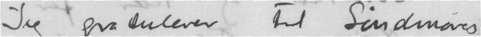Jeg gratulerer til Søndmøres
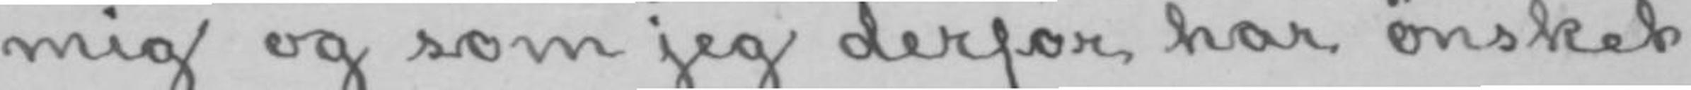mig og som jeg derfor har ønsket
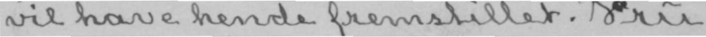vil have hende fremstillet. Fru
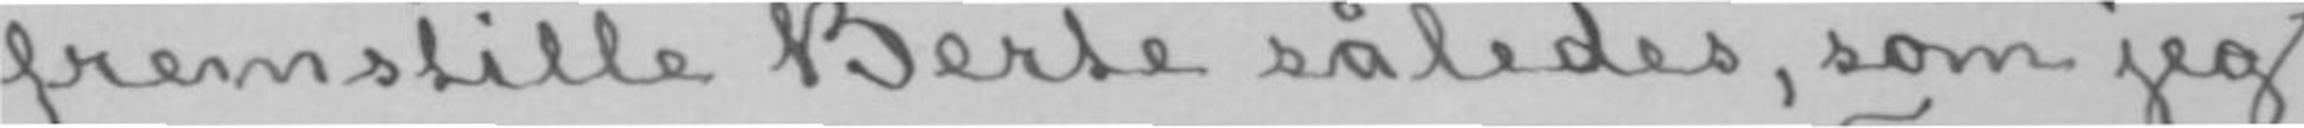fremstille Berte således, som jeg
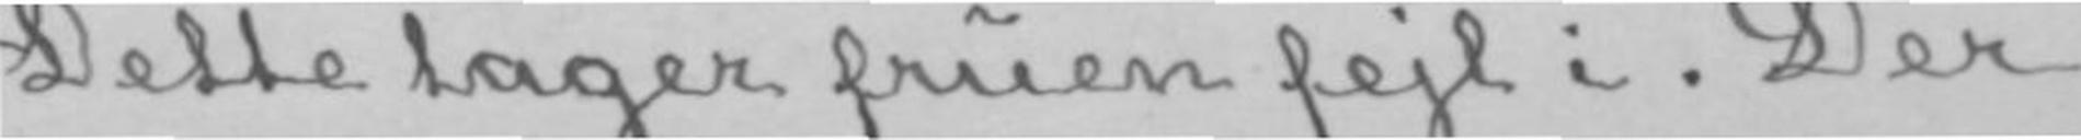Dette tager fruen fejl i. Der
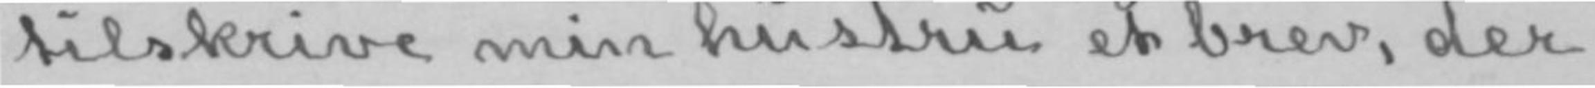tilskrive min hustru et brev, der
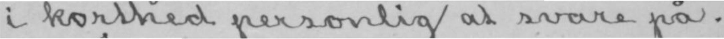i korthed personlig at svare på.
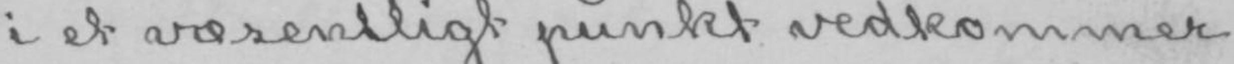i et væsentligt punkt vedkommer
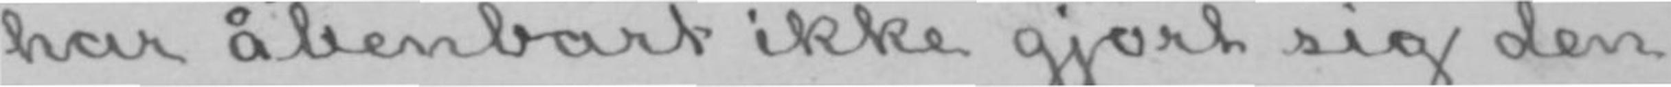har åbenbart ikke gjort sig den
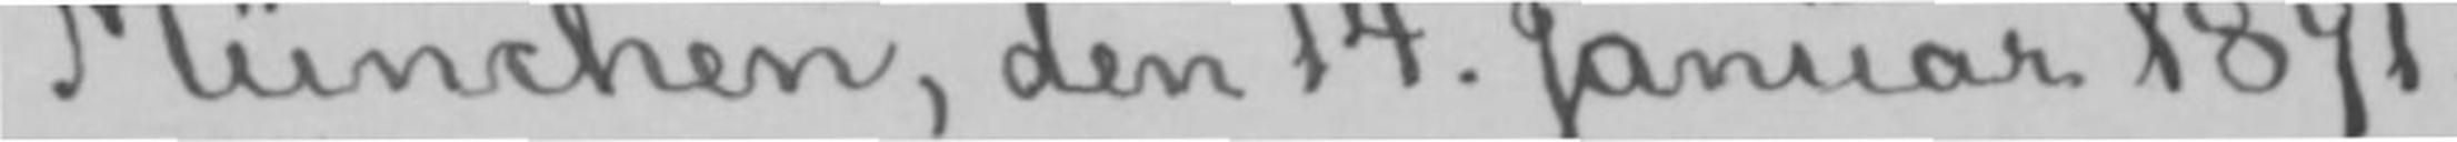München, den 14. Januar 1891.
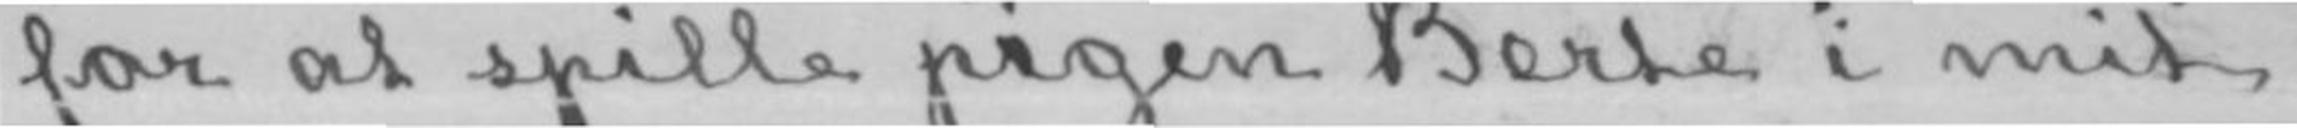for at spille pigen Berte i mit
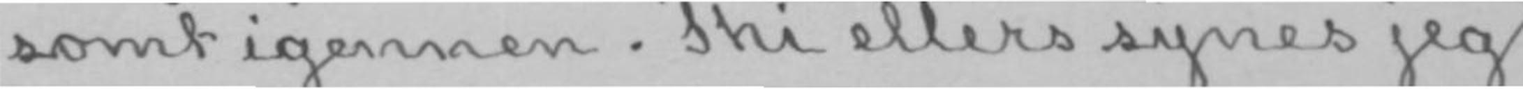somt igennen. Thi ellers synes jeg
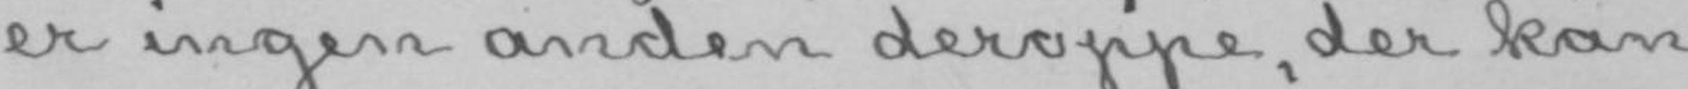er ingen anden deroppe, der kan
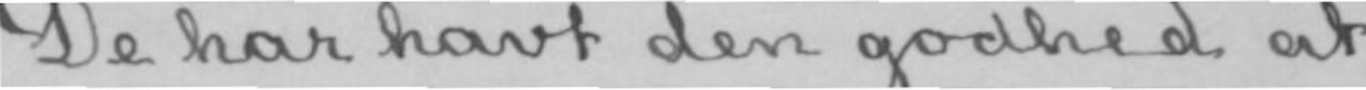De har havt den godhed at
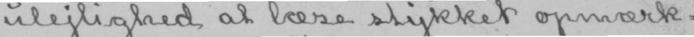ulejlighed at læse stykket opmærk-
